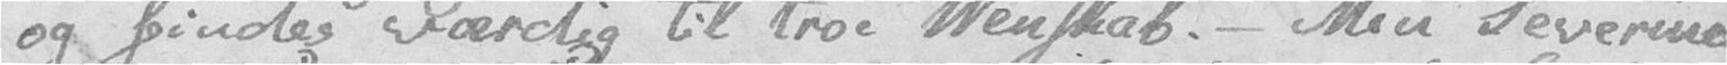og findes værdig til troe Venskab. – Min Severine
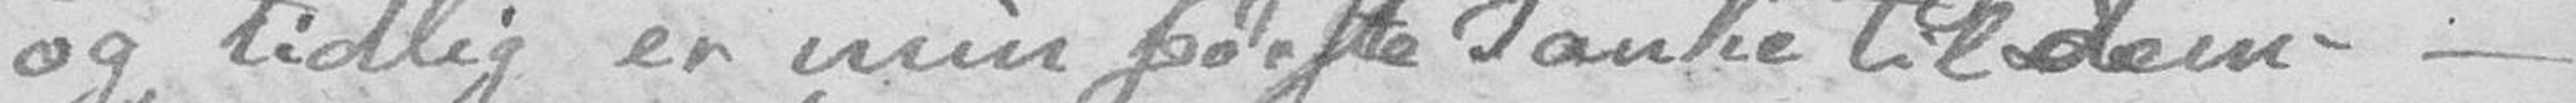og tidlig er min første Tanke til dem. –
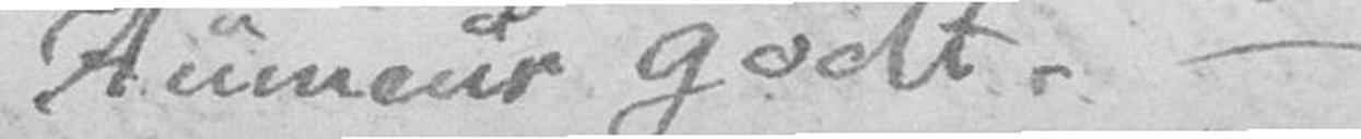Humeur godt. –
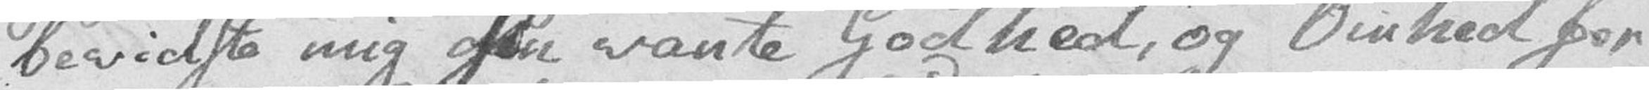bevidste mig sin vante Godhed, og Ømhed for
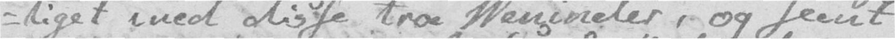-tiget med disse troe Veninder, og seent
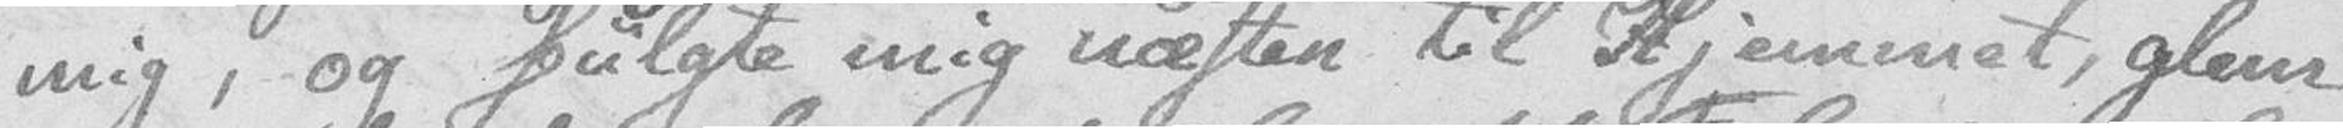mig, og fulgte mig næsten til Hjemmet, glem
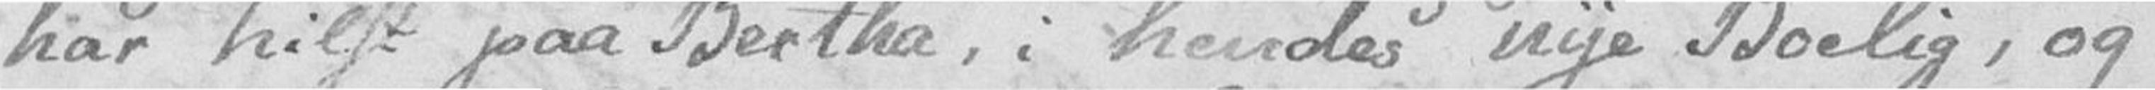har hilst paa Bertha, i hendes nye Boelig, og
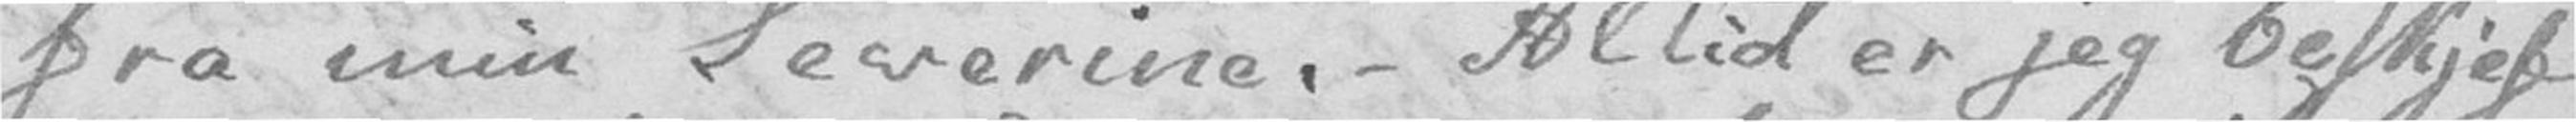fra min Severine. – Altid er jeg beskjef
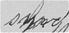synd
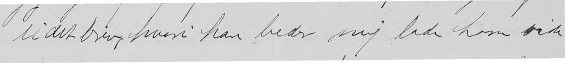lidet brev, hvori han beder mig lade ham vite
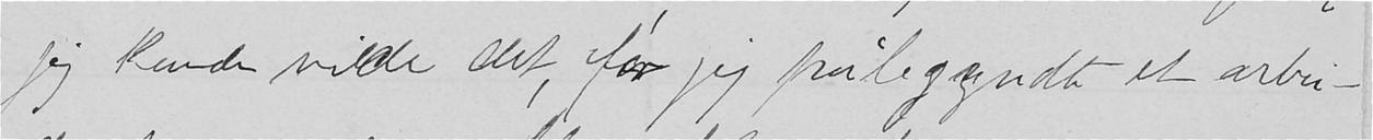jeg kunde vilde det, før jeg påbegyndte et arbei-
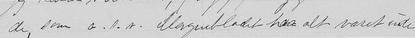de, som o.s.v. Morgenbladet har alt været ude
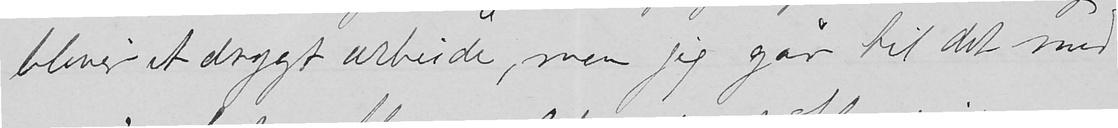bliver et drygt arbeide, men jeg går til det med
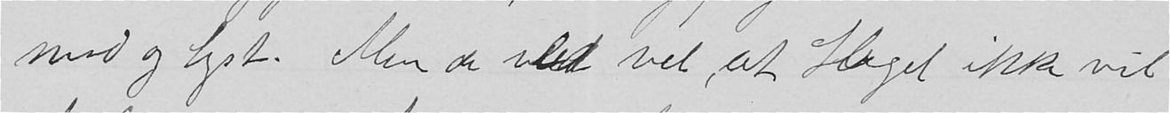mod og lyst. Men de ved vel, at Hegel ikke vil
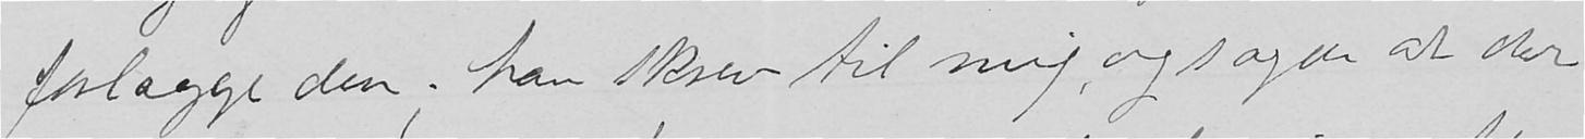forlægge den; han skrev til mig og sagde at der
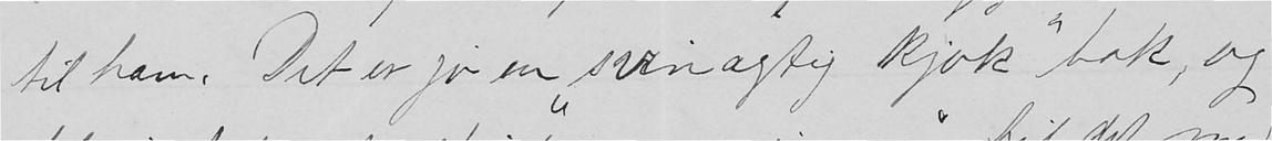til ham. Det er jo en "svinagtig kjok" bok, og
